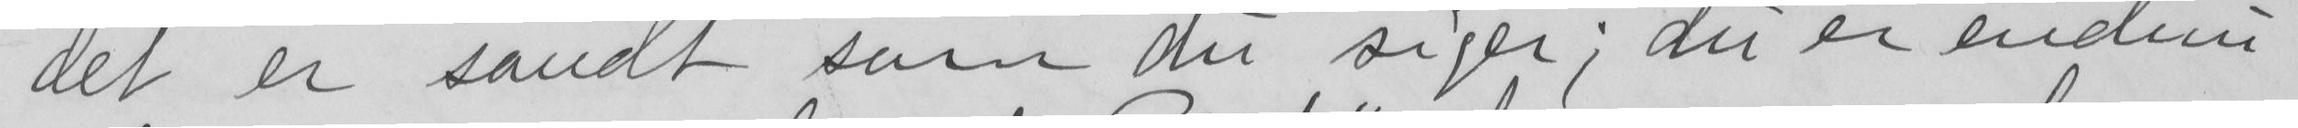det er sandt som du siger; du er endnu
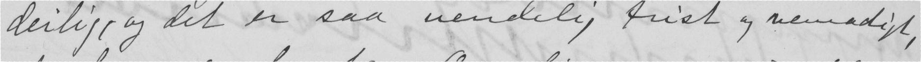deilig, og det er saa uendelig trist og vemodigt,
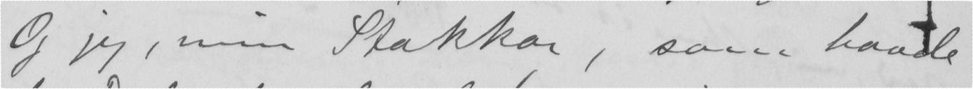Og jeg, min Stakkar, som havde
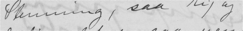Stemning, saa rig og
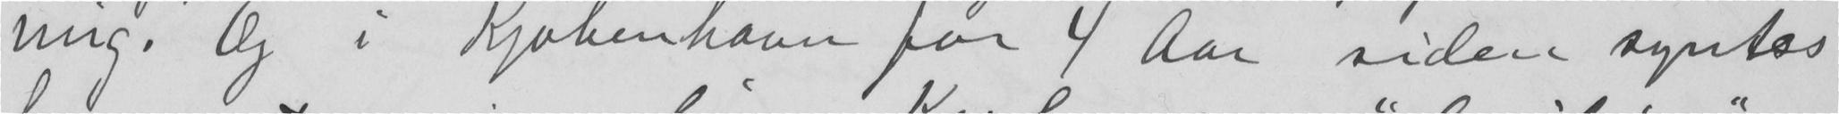mig. Og i Kjøbenhavn for 4 Aar siden syntes
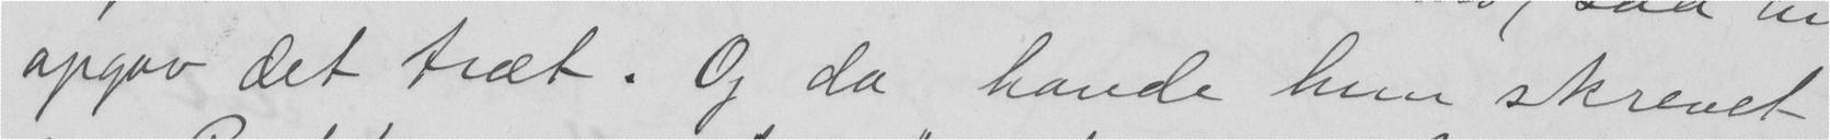opgav det træt. Og da havde hun skrevet
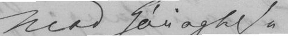Med høiagtigt
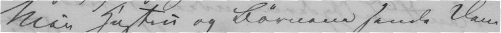Min Hustru og Børnene sende Dem
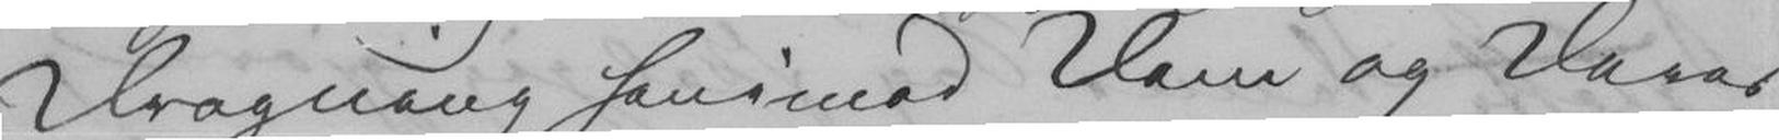Dragning henimod Dem og Deres
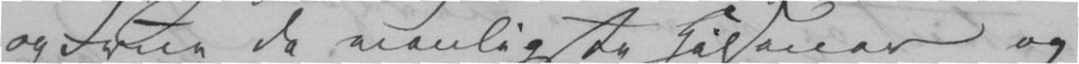og frue de venligste hilsener og
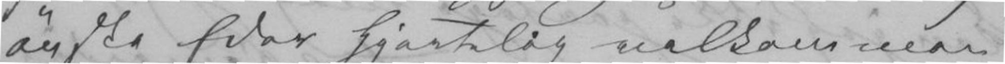ønske Eder hjærtelig velkommen
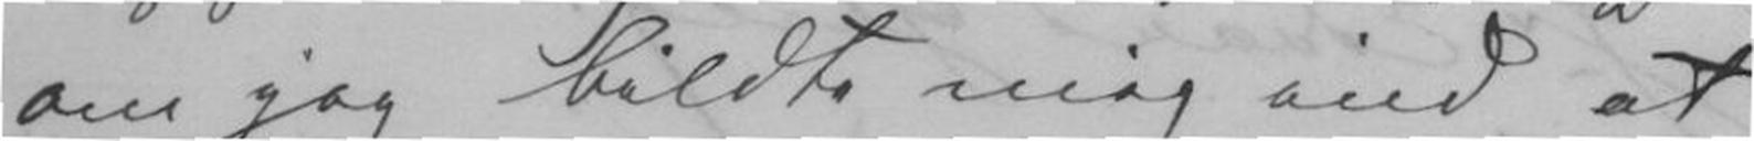om jeg bildte mig ind at
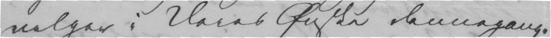velger i Deres Ønske dennegang.
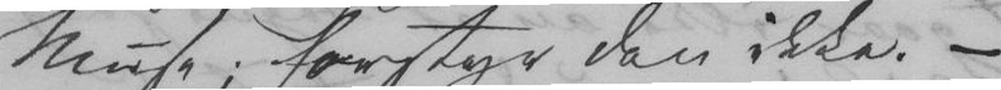Muse.; forstyr den ikke. -
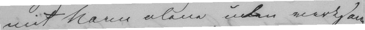mit Navn alene uden verdigsom
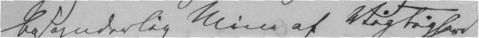besynderlig Mine af Vigtighed
